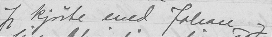Jeg kjørte med Johan og
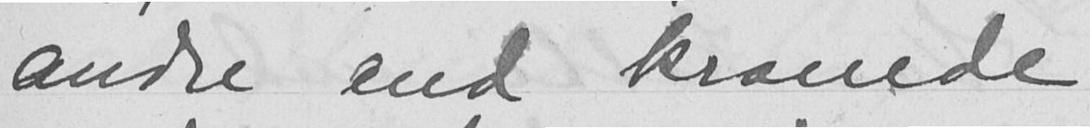andre end kronede
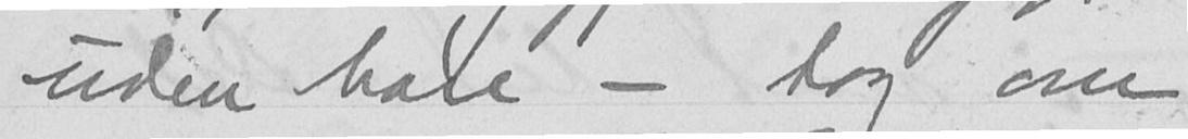uden hare - tog om
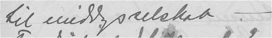til middagsselskab -
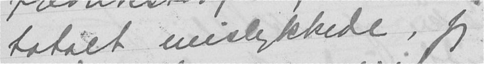totalt mislykkede, jeg
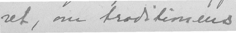ret, om traditionens
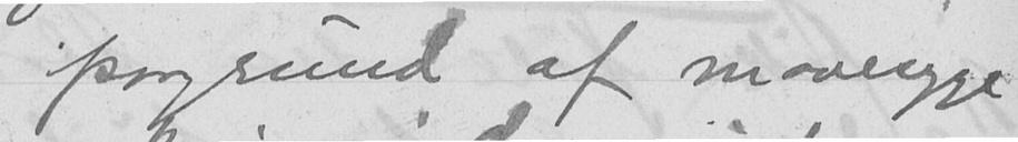paagrund af mavesyge.
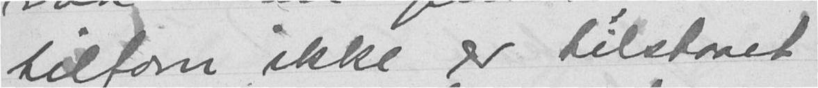tilforn ikke er tilstaaet
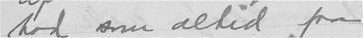bad som altid paa
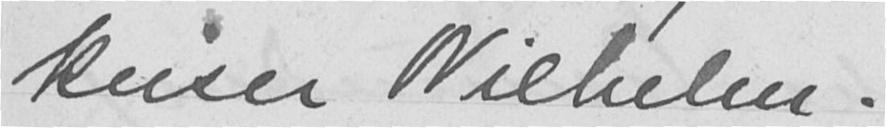keiser Wilhelm.
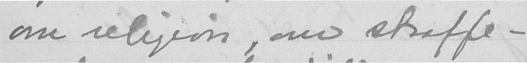om religion, om straffe-
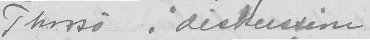Thorsø. diskussion
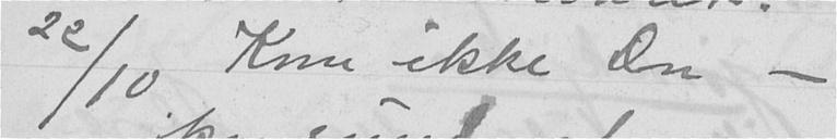22/10 Kom ikke Don -
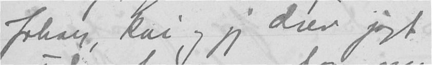Johan, Kai og jeg drev jagt
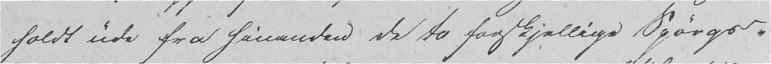holdt ude fra hinanden de to forskjellige Spørgs-
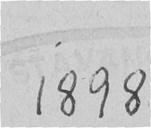1898
